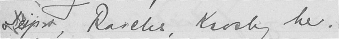Seips, Raschs, Krosby her.
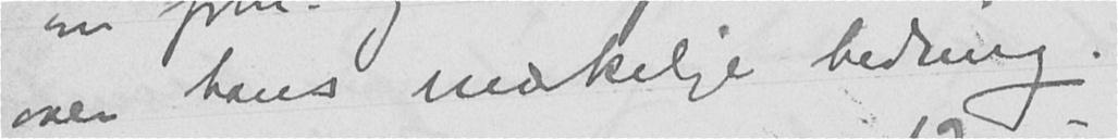over hans makelige bedring.
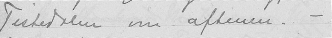Tistedalen om aftenen. -
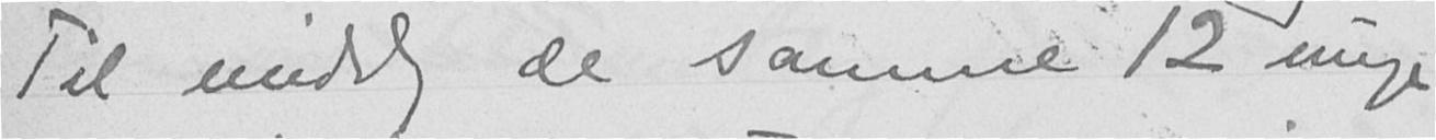Til middag de samme 12 unge
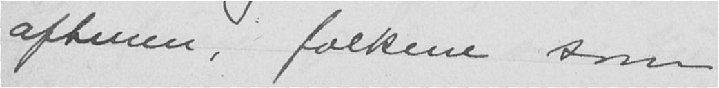aftenen, folkene som
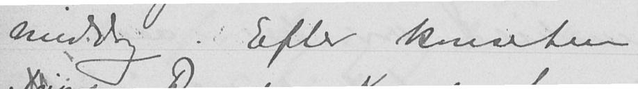middag. Efter konserten
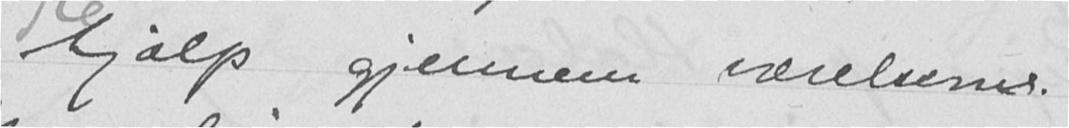hjælp gjennem værelsene.
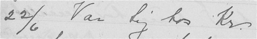22/6 Var Sig hos Kr.
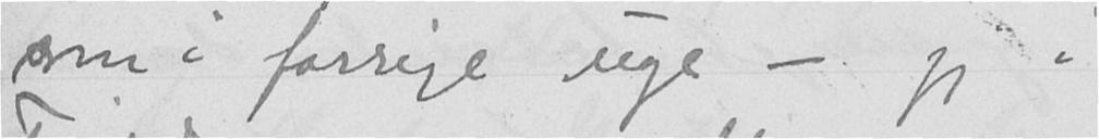som i forrige uge - jeg i
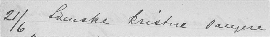21/6 Svenske kristne sangere
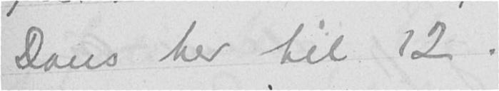Dans her til 12.
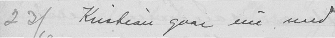23/6 Kristian gaar nu med
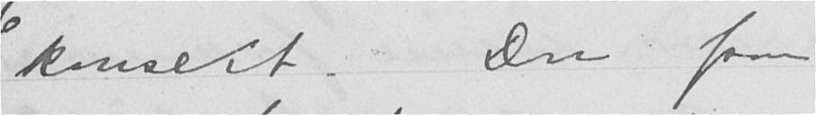konsert. Don paa
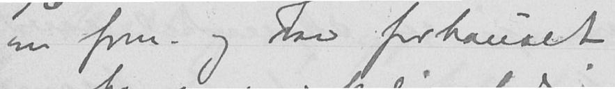om form. og var forbauset
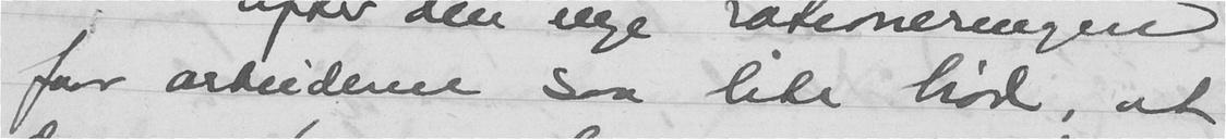faar arbeideren saa lite brød, at
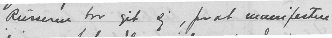Russerne har git sig, for at manifestere
